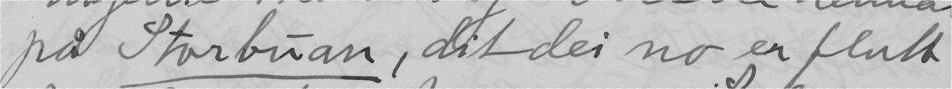på Storbuan, dit dei no er flutt
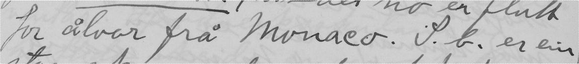for ålvor frå Monaco. S.b. er ein
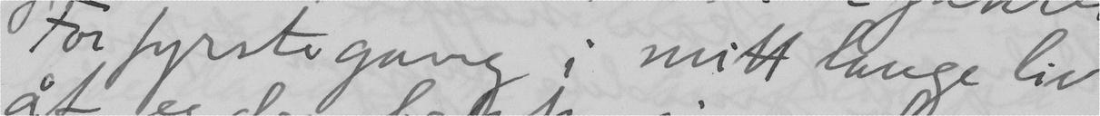For fyrste gang i mitt lange liv
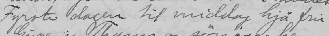Fyrste dagen til middag hjå fru
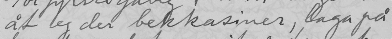åt eg der bekkasiner, laga på
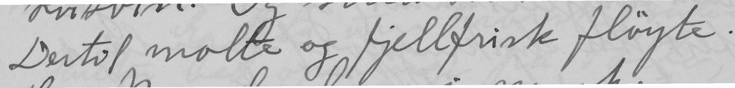Dertil molte og fjellfrisk flöyte.
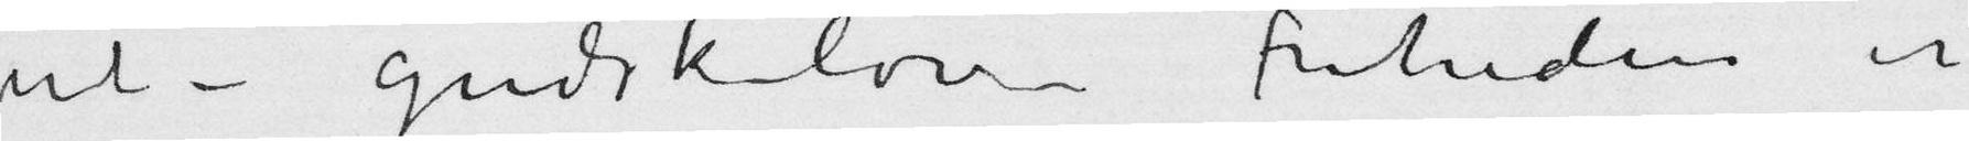ut – gudskelov – Friheden er
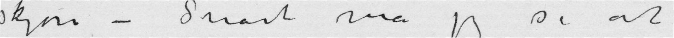skjøn – Snart må jeg se at
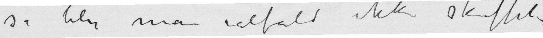så blir man ialfald ikke skuffet
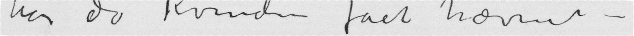har da Kvinden fået hævnet –
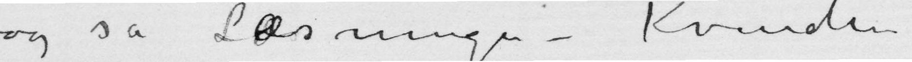og så læsningen – Kvinden
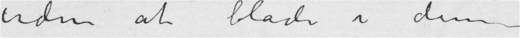uden at blade i dem
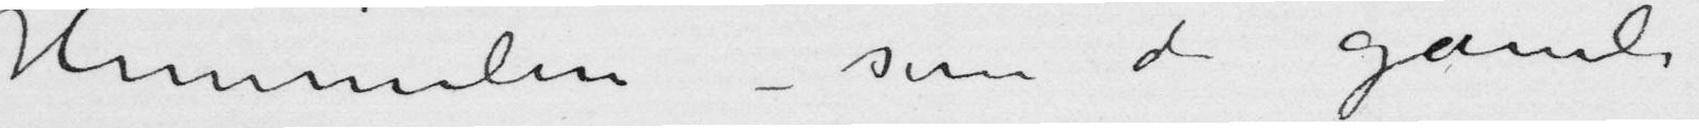Himmelen – som de gamle
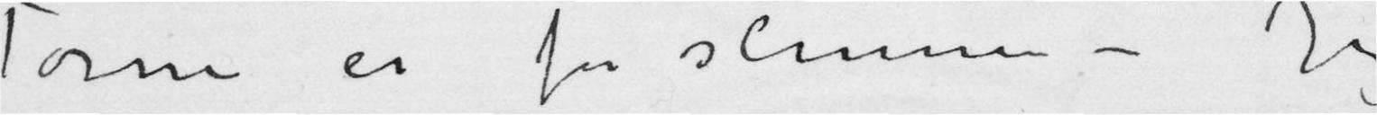Torne er for slemme – Jeg
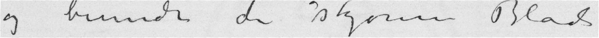og beundre de skjønne Blade
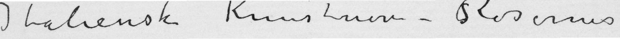italienske Kunstnere –	Rosernes
